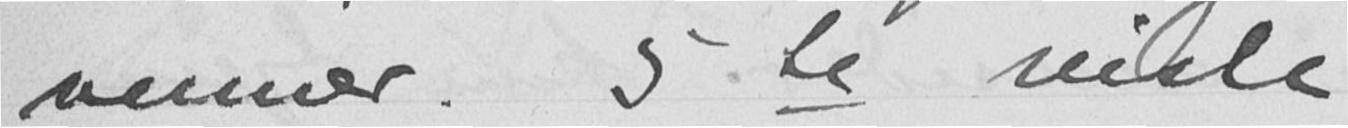venner. 5te reiste
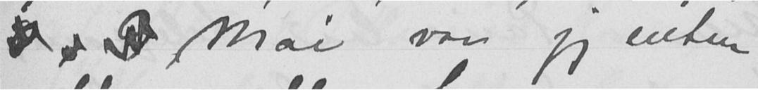8, 9 Mai var jeg enten
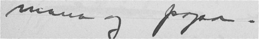mama og papa.
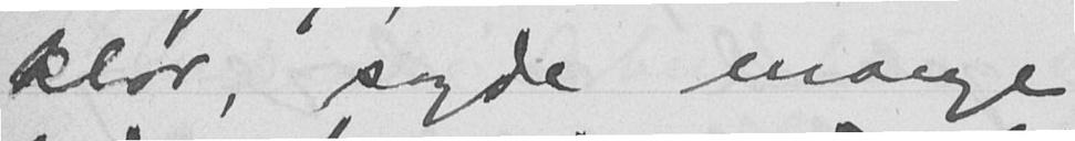klar, sagde mange
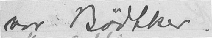var Bødtker.
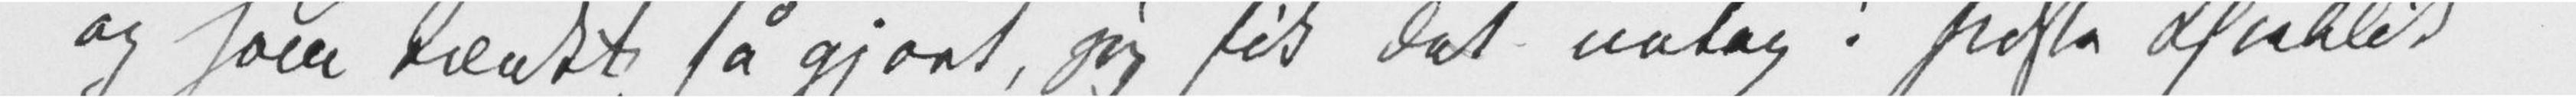og som tænkt så gjort, jeg fik det netop i sidste Øieblik
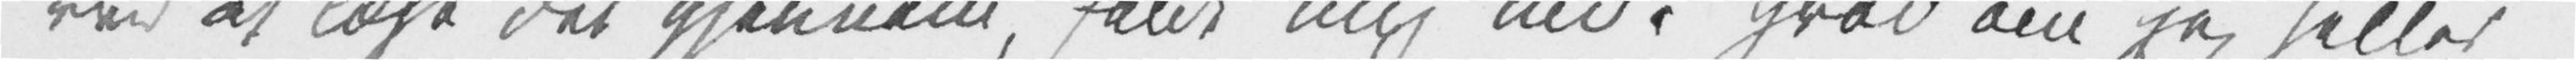ved at læse det gjennem, faldt mig ind: hvad om jeg heller
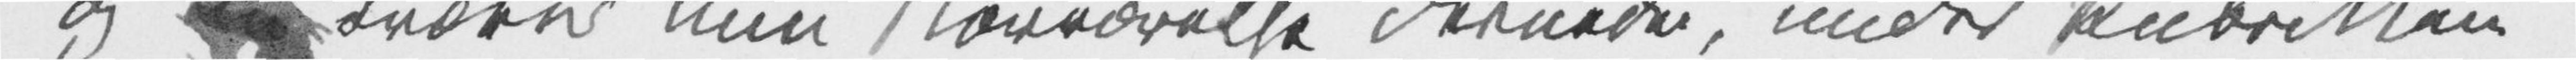og nu kræver min Nærværelse dernede, under Rubrikken
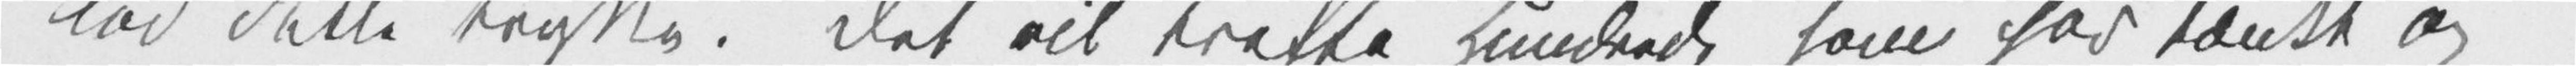lod dette trykke? Det vil treffe Hundrede som har tænkt og
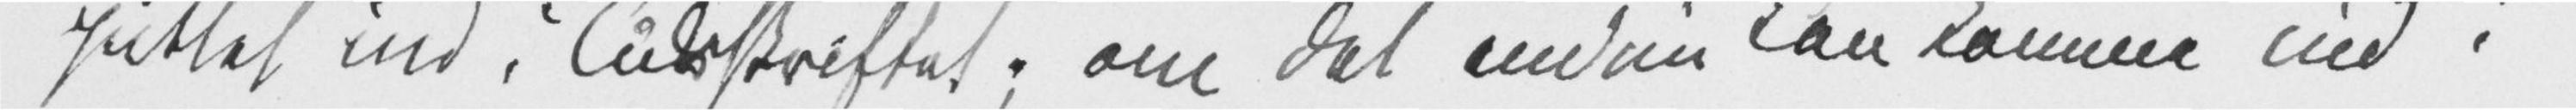puttet ind i Tidsskriftet; om det endnu kan komme ind i
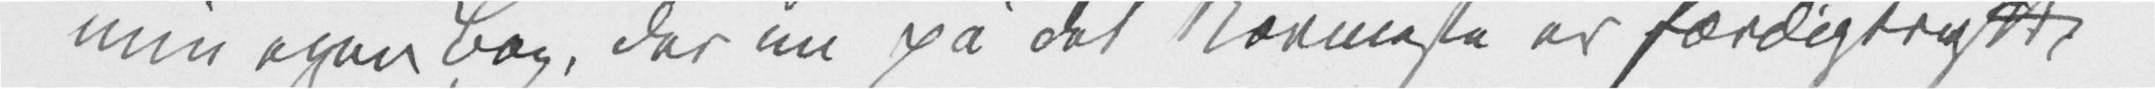min egen Bog, der nu på det Nærmeste er færdigtrykt
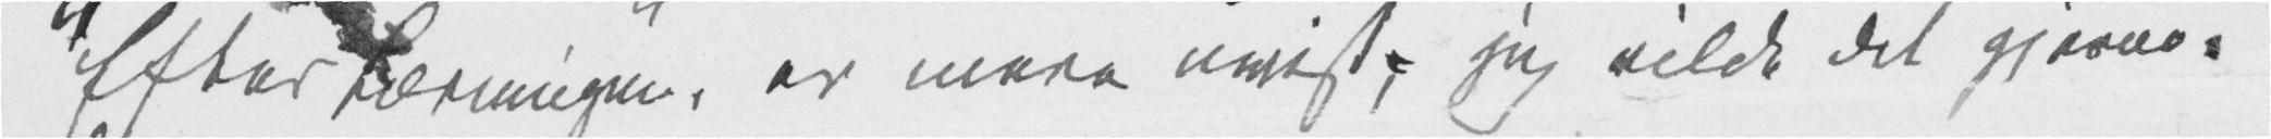«Efter Læsningen», er mere uvist, jeg vilde det gjerne.
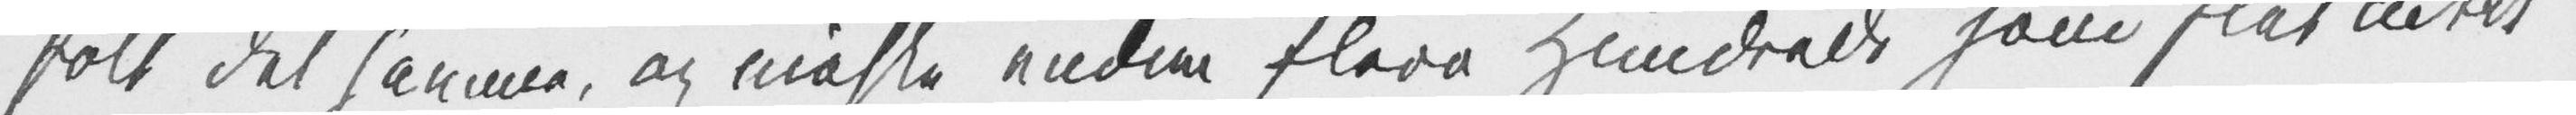følt det samme, og måske endnu flere Hundrede som slet intet
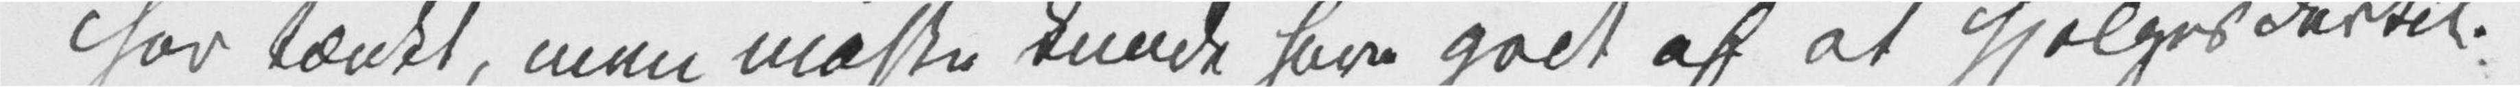har tænkt, men måske kunde have godt af at hjelpes dertil,
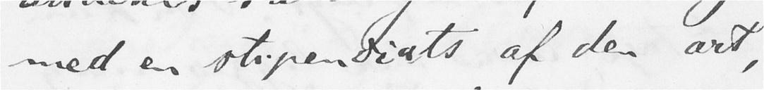med en stipendiats af den art,
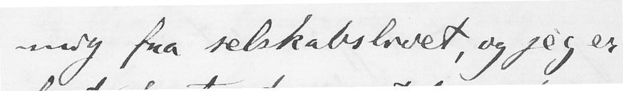mig fra selskabslivet, og jeg er
